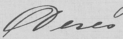Deres
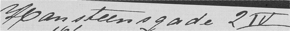Hansteensgade 2 IV
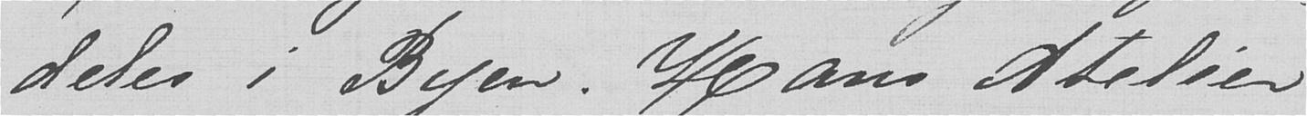deles i Byen. Hans Atelier
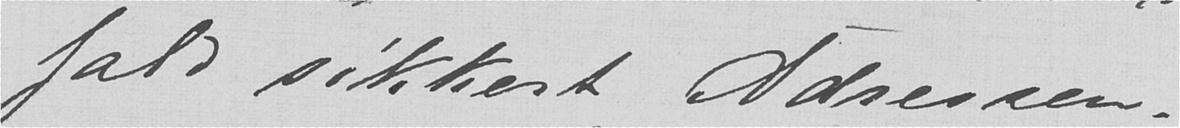fald sikkert Adressen.
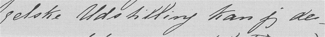gelske Udstilling kan jeg des-
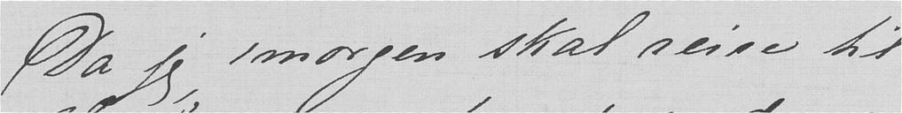Da jeg imorgen skal reise til
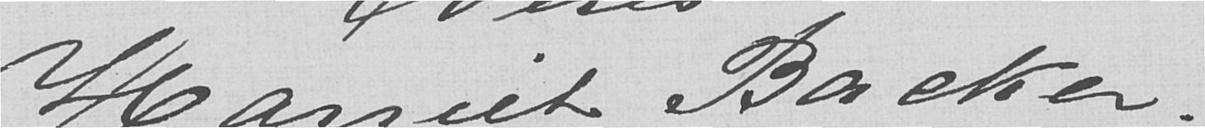Harriet Backer.
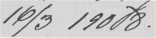16/3 1908.
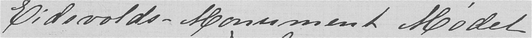Eidsvolds-Monument Mødet
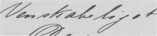Venskabeligst
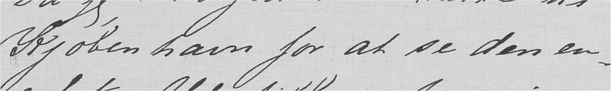Kjøbenhavn for at se den en-
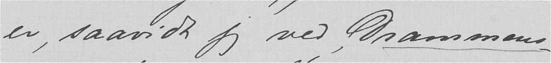er, saavidt jeg ved, Drammens-
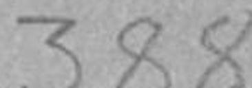388
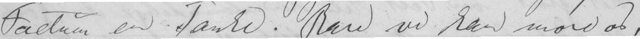Factum en Tanke. Bare vi kan more os,
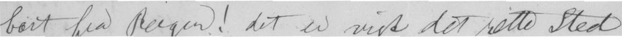bort fra Bergen! det er vist det rette Sted
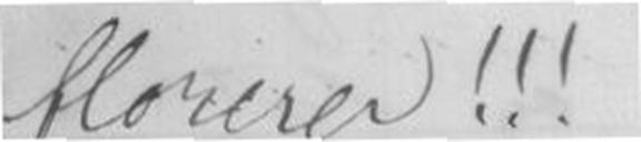florerer!!!
